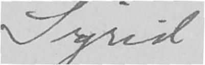Sigrid
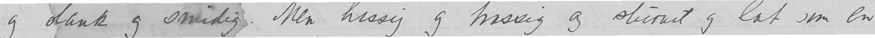og slank og smidig. Men hissig og trassig og slurvet og lat som en
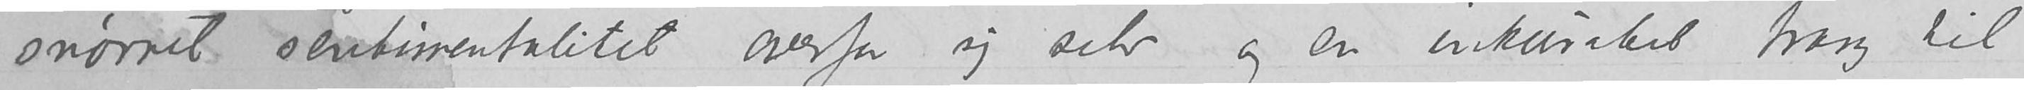snørret sentimentalitet overfor sig selv og en inkurabel trang til
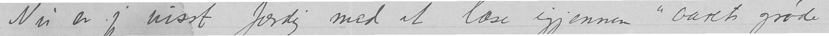Nu er jeg visst færdig med at læse igjennem «aarets grøde»
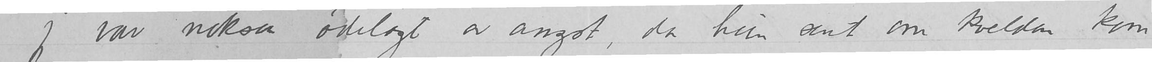og jeg var noksaa ødelagt av angst, da hun sent om kvelden kom
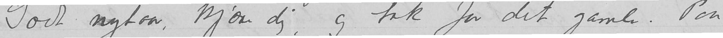Godt nytaar, kjære dig, og tak for det gamle. Paa
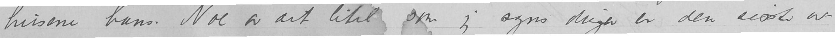ehusene hans. Noe av det litet som jeg syns duger er den sisste av
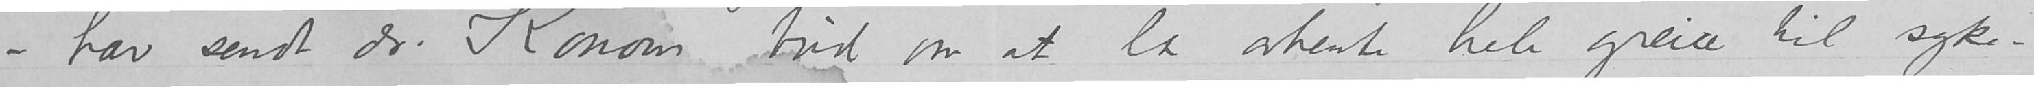–har sendt dr. Konow bud om at la avhente hele greia til syk
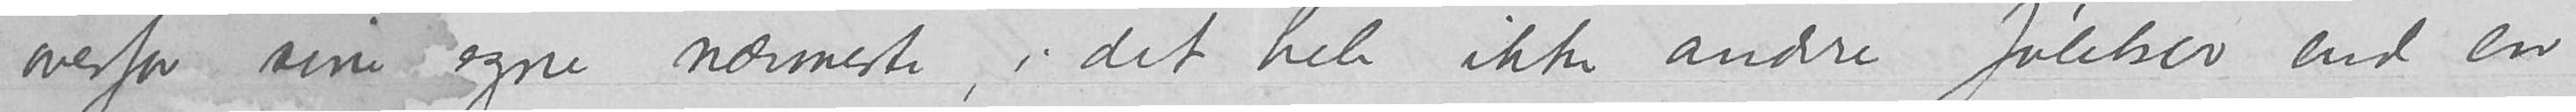overfor sine egne nærmeste, i det hele ikke andre følelser end en
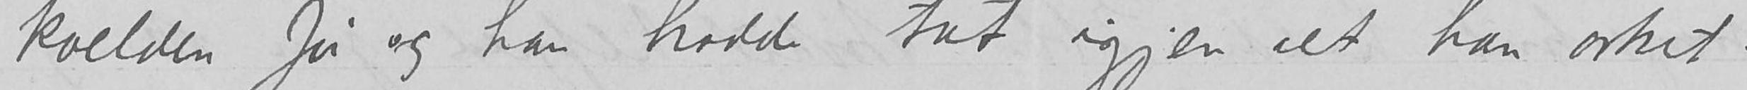kvelden før og han hadde tat igjen alt han orket.
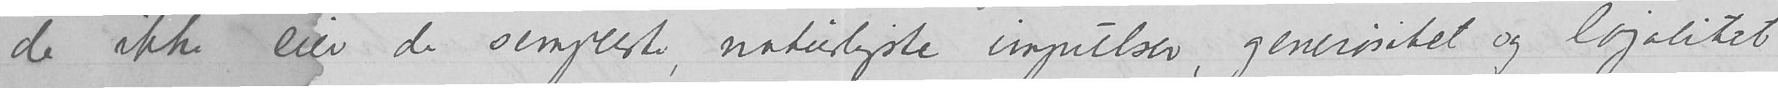de ikke eier de simpleste, naturligste impulser, generøsitet og lojalitet
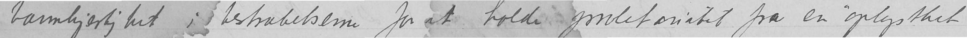barmhjertighet i bestræbelserne for at holde proletariatet fra en «oplysthet»
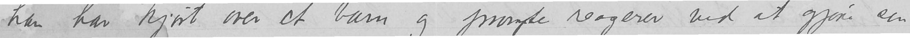han har kjørt over et barn og prompte reagerer ved at gjøre sin
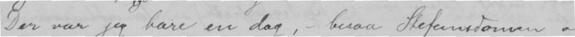Der var jeg bare en dag, - besaa Stefunsdomen og
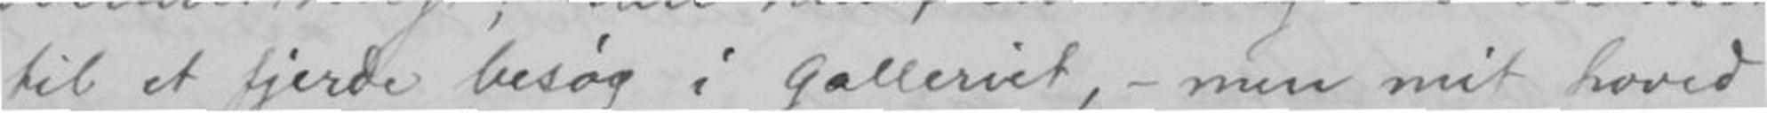til et fjerde besøg i galleriet,- men mit hoved
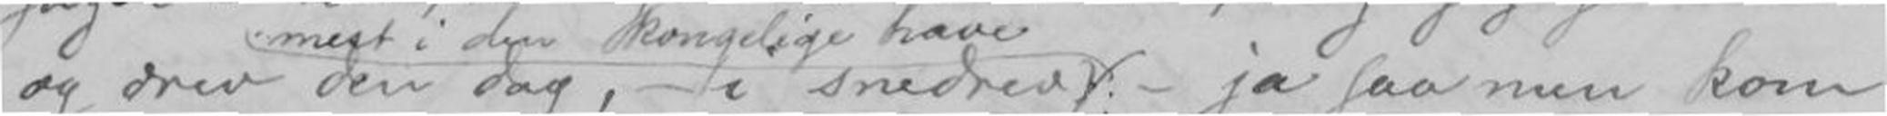og drev den dag, - i snedrev; - ja saa men kom
